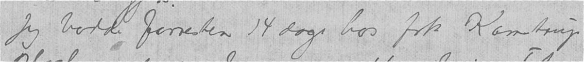Jeg bodde forresten 14 dage hos frk Kamstrup
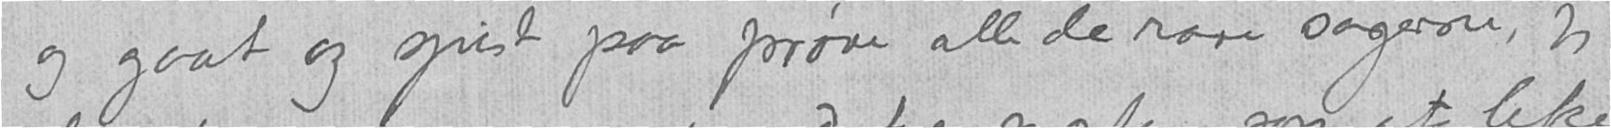og gaat og spist paa prøve alle de rare sagerne, jeg
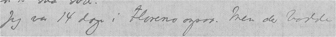Jeg var 14 dage i Florens ogsaa. Men der bodde
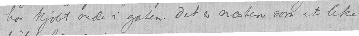har kjøbt nede i gaten. Det er næsten som at leke
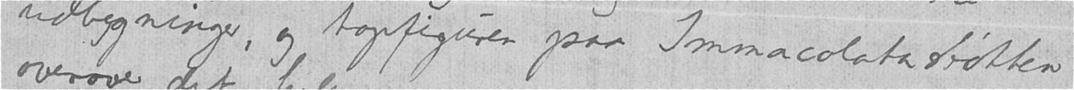udbygninger, og topfiguren paa Immacolatastøtten
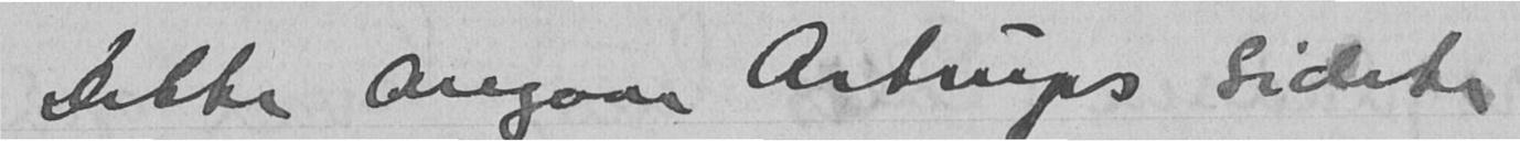Dette angaar Astrups sidste
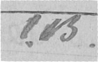113
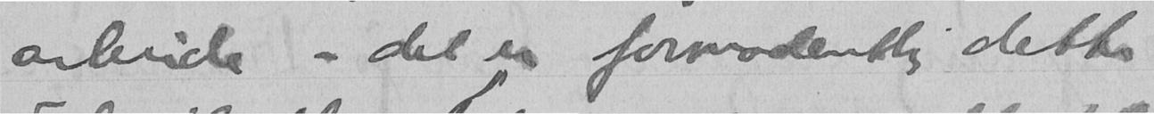arbeide - det er formodentlig dette
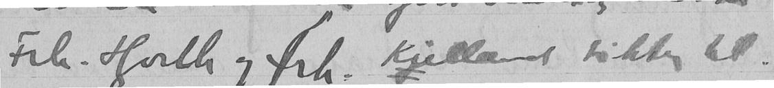Frk. Hjorth og frl. Kielland sikter til.
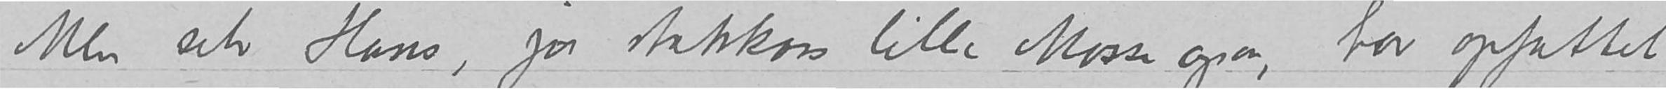Men selv Hans, ja stakkars lille Mosse ogsaa, har opfattet
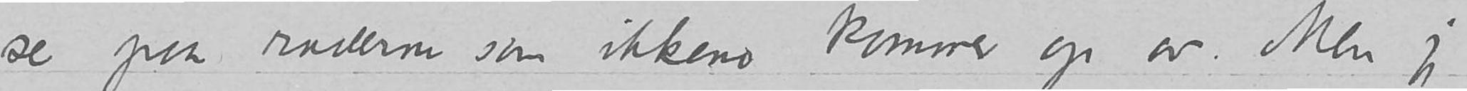se paa raderne som ikkeno kommer op av. Men jeg
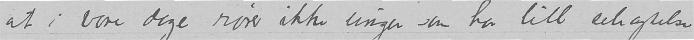at i vore dage rører ikke unger som har litt selvagtelse
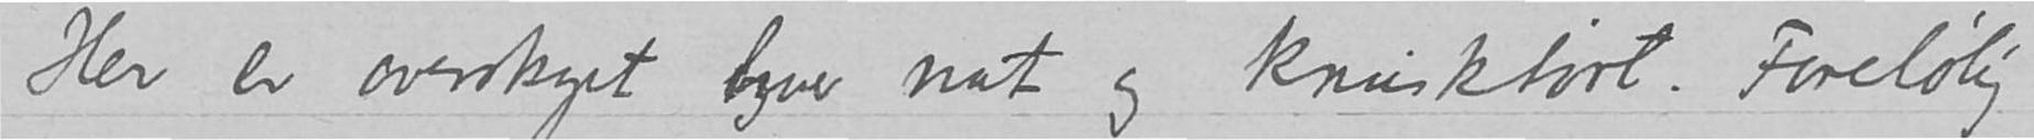Her er overskyet hver nat og knusktørt.
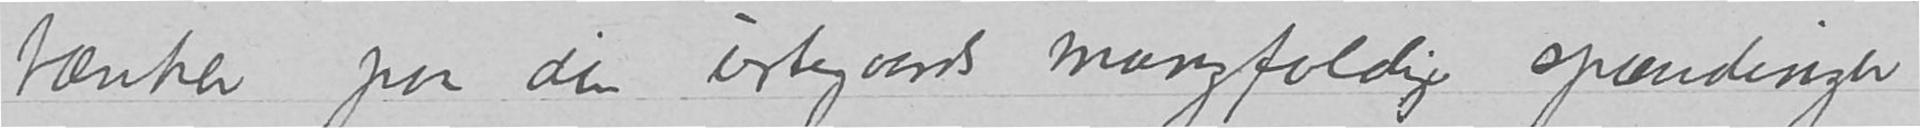tænker paa din urtegaards mangfoldige spændinger
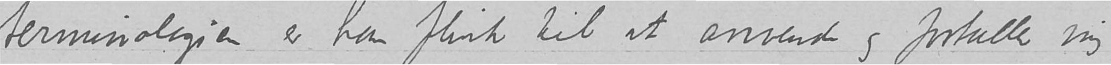terminologien er han flink til at anvende og forteæller mig
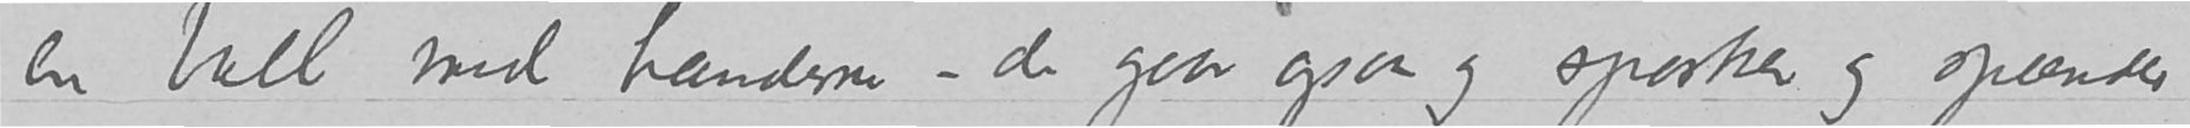en ball med hænderne - de gaar ogsaa og sparker og spænder
